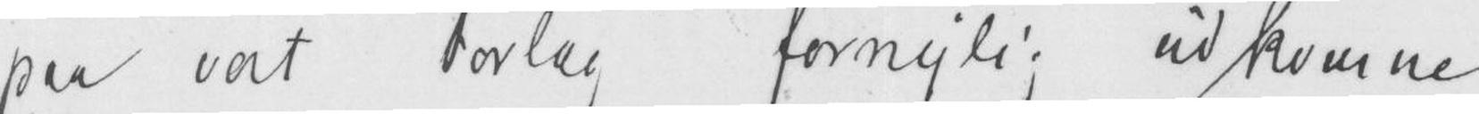paa vort Forlag fornylig udkomne
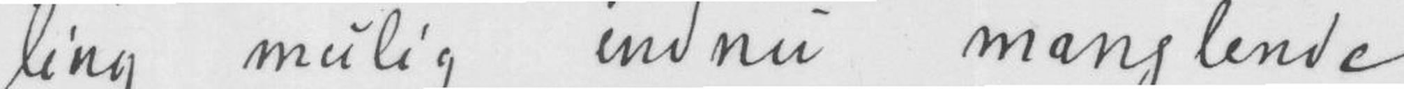ling mulig endnu manglende
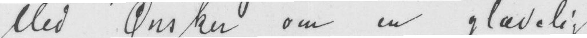Med Ønsker om en glædelig
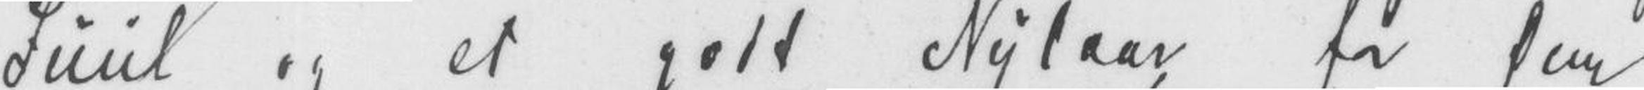Juul og et godt Nytaar fra Dem
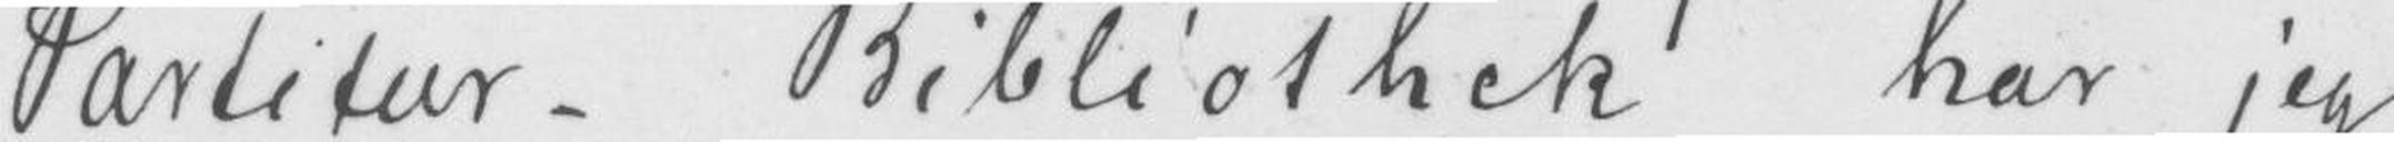Partitur- Bibliothek har jeg
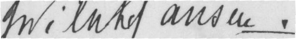Wilhelm Hansen.
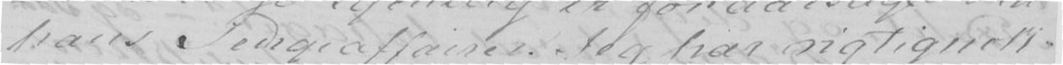hans Pengeaffairer. Jeg har rigtignok
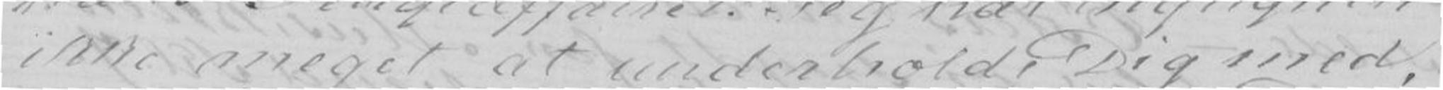ikke meget at underholde Dig med,
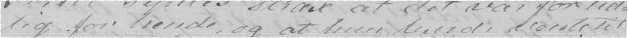lig for hende, og at hun burde Vente til
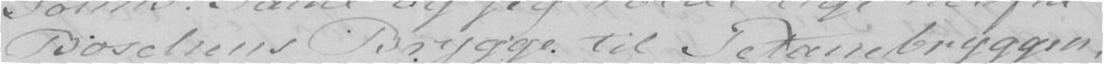Bøschens Brugge til Petanebryggen
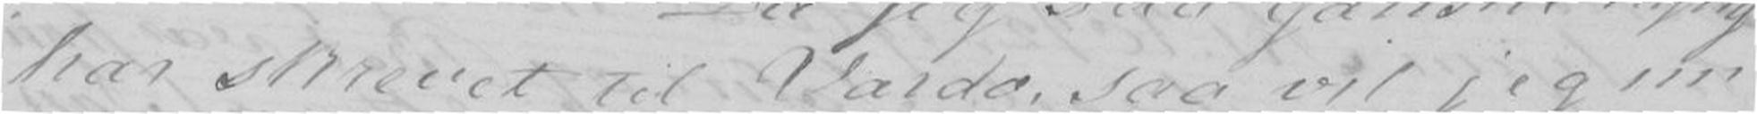har skrevet til Vardo, saa vil jeg nu
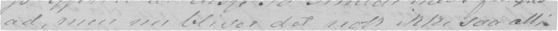ad, men nu bliver det nok ikke saa alli-
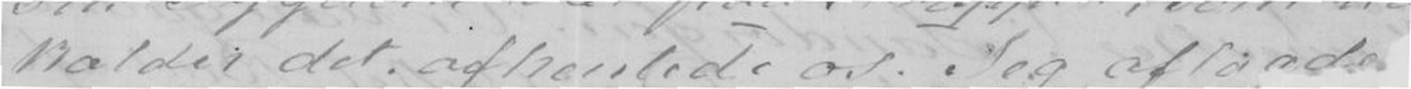kalder der afhentede os. Jeg aflaad
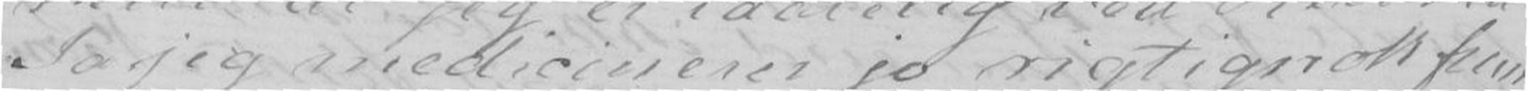Ja jeg medicinerer jo rigtignok frem-
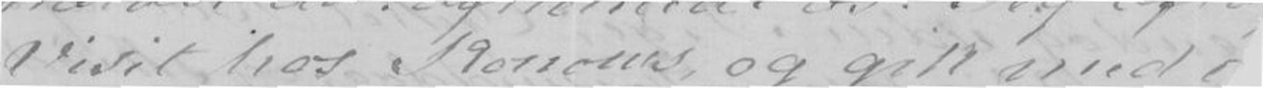Viisit hos Konows, og gik med i
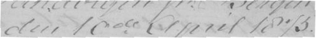den 10de April 1875.
